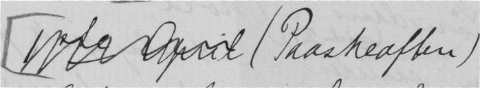(Paaskeaften)
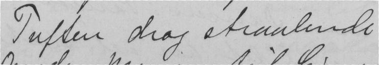Tuften drog straalende
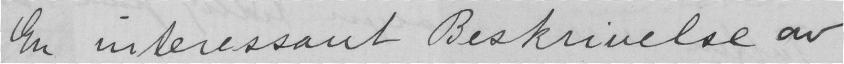En interessant Beskrivelse av
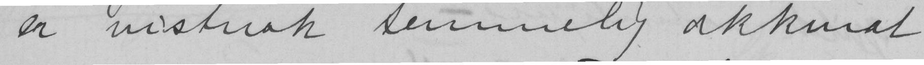er vistnok temmelig akkurat
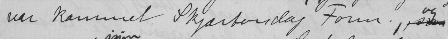var kommet Skjærtørsdag Form.,
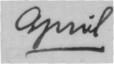april
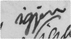igjen
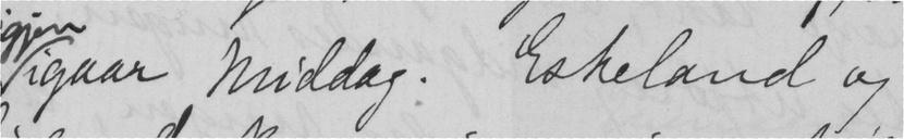igaar Middag. Eskeland og
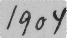1904
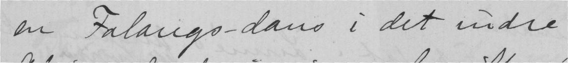en Falangs-dans i det indre
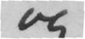og
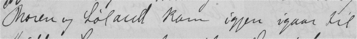Moren og Løland kom igjen igaar til
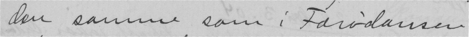den samme som i Færødansen
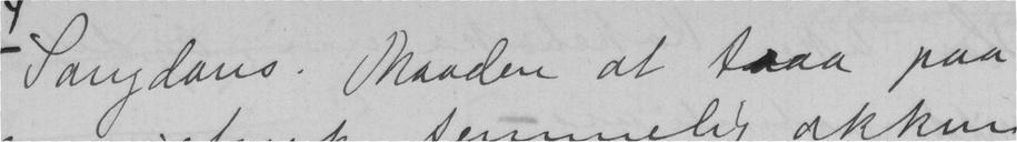Sangdans. Maaden at traa paa
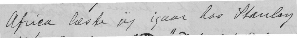Africa læste jeg igaar hos Stanley
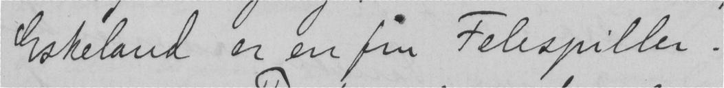Eskeland er en fin Felespiller.
